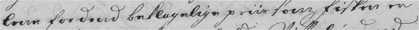lene for dend beklagelige priis som Fisken er
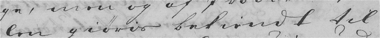len giøres bekiendt til
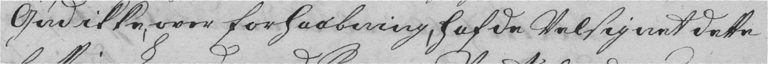Gud ikke, over forhaabning, hafde Velsignet dette
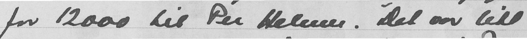for 12000 til Per Helmer. Det var litt
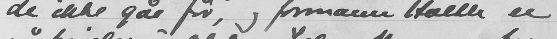de ikke går før, og formann Holth er
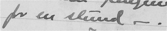for en stund - .
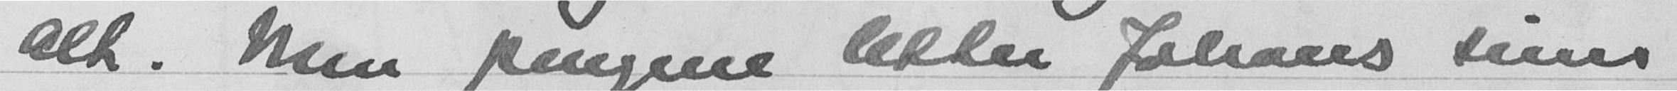alt. Men pengene letter Johans sinn
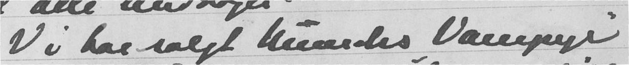Vi har solgt Munchs Vampyr
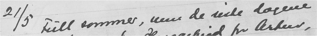21/5 Full sommer, men de siste dagene
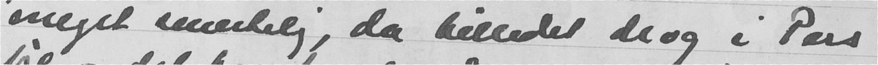meget smertelig, da billedet drog i Pars
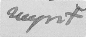mynt.
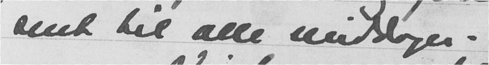sent til alle middager.
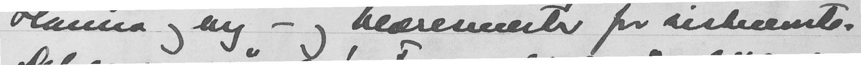Hanna og mig - og blæresmerter for sistnevnte.
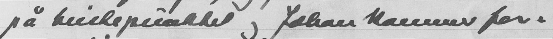på bristepunktet og Johan kommer for-
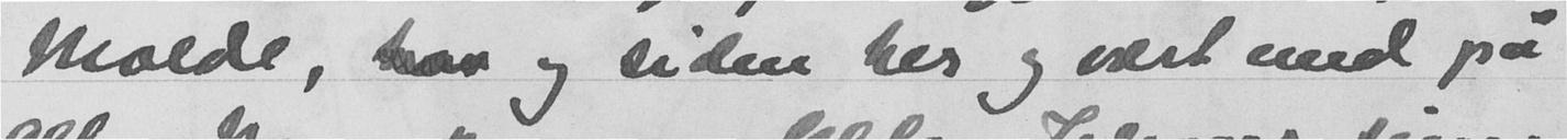Molde,  og siden her og vært med på
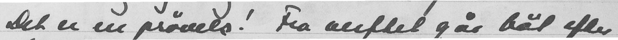Det er en prøvelse. Fra verftet går båt efter
